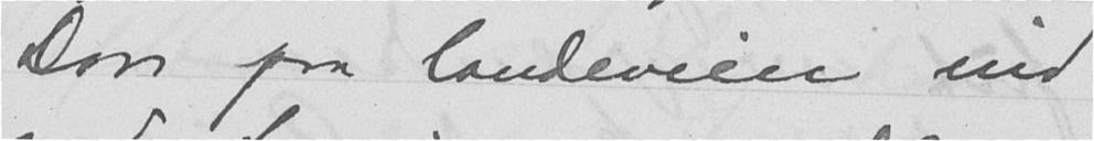Don paa landeveien ind
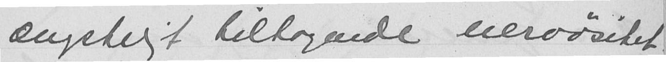ængstligt tiltagende nervøsitet.
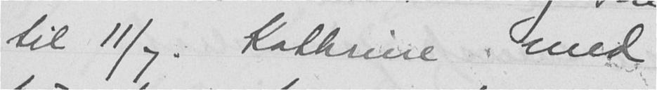til 11/7. Kathrine med
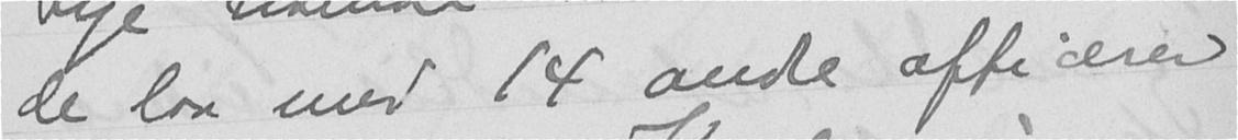de laa med 14 andre officerer
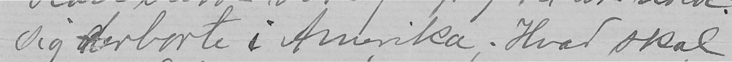sig derborte i Amerika. Hvad skal
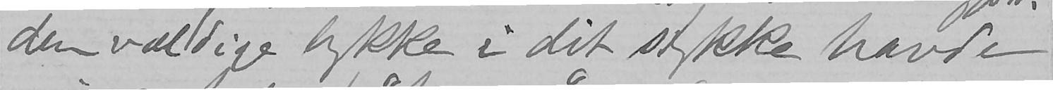den vældige lykke i dit stykke havde
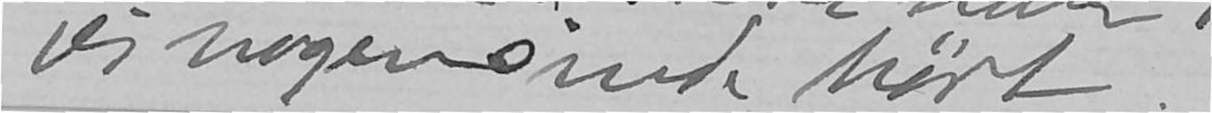jeg nogensinde hørt.
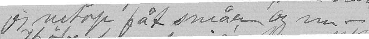jeg netop fåt småen og nu -
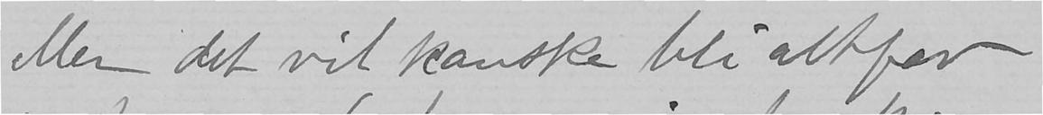Men det vil kanske bli altfor
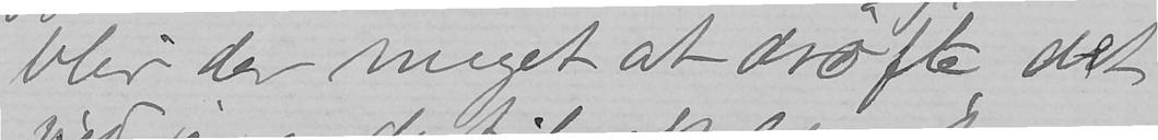blir der meget at drøfte, det
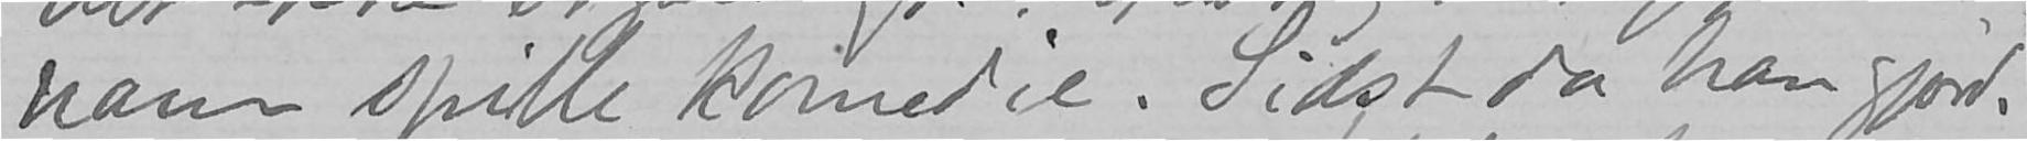ham spille komedie. Sidst da han gjorde
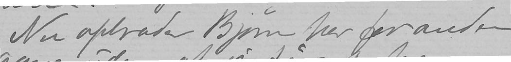Nu optræder Bjørn her for anden
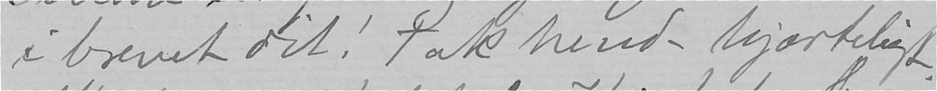i brevet dit! Tak hende hjærteligt.
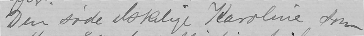Den søde elskelige Karoline som
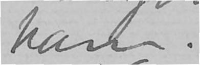han.
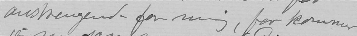anstrengende for mig, for kommer
